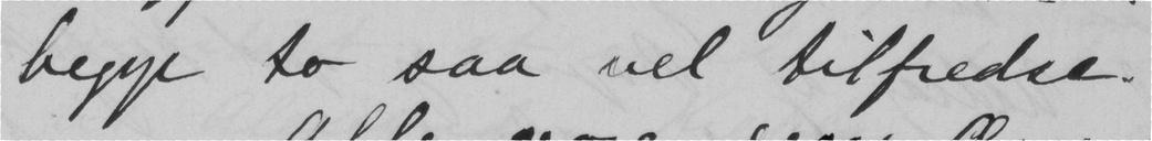begge to saa vel tilfredse.
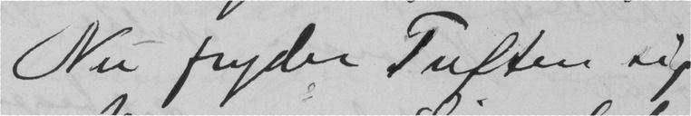Nu fryder Tuften sig
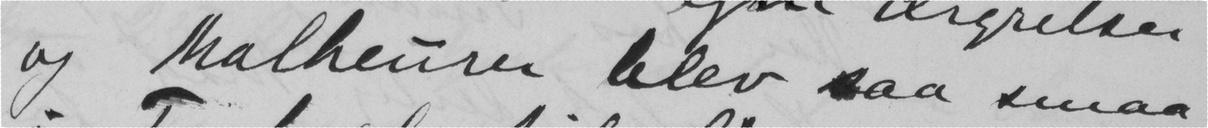og Malheurer blev saa smaa
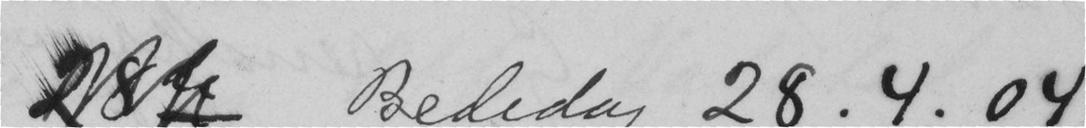Bededag 28.4.04
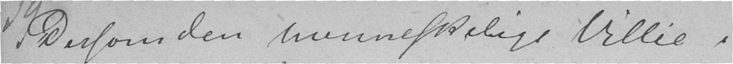Dersom den menneskelige Villie i
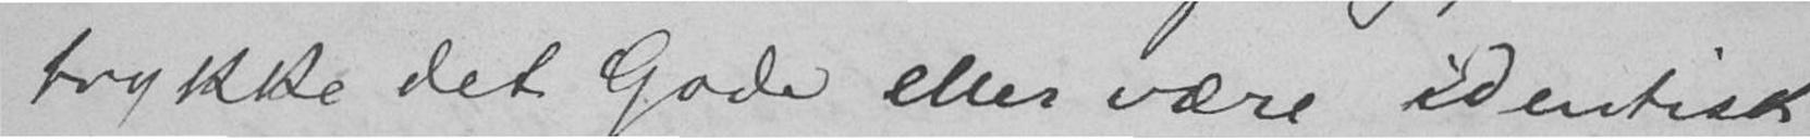trykke det Gode eller være identisk
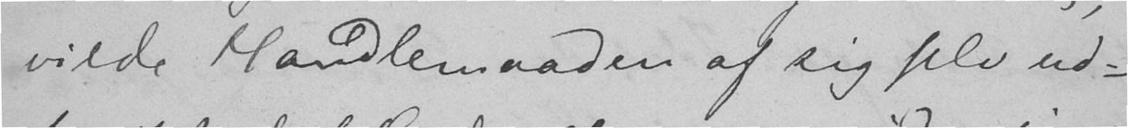vilde Handlemaaden af sig selv ud-
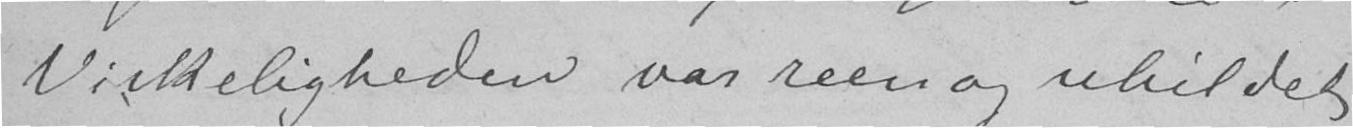Virkeligheden var reen og uhildet,
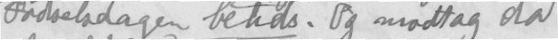Fødselsdagen betids. Og modtag da
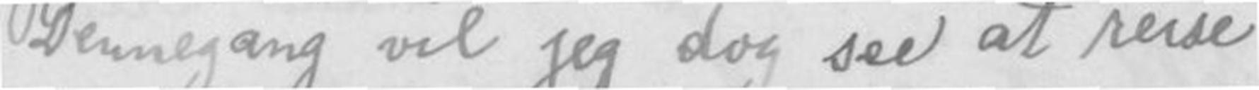Dennegang vil jeg dog see at reise
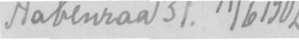Aabenraa 31. 11/6 1902
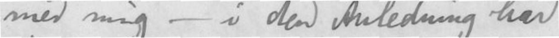med mig - i den Anledning har
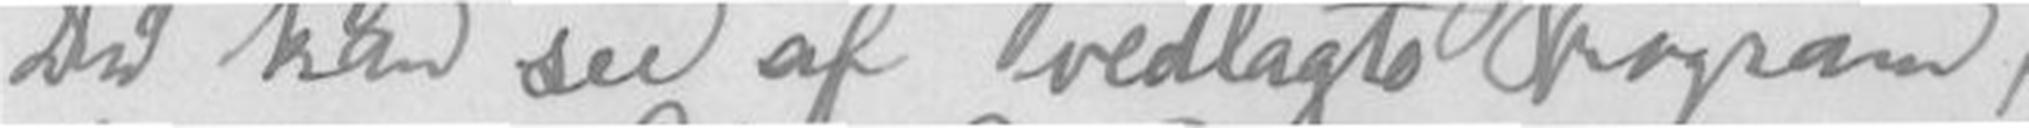Du kan see af vedlagte Program,
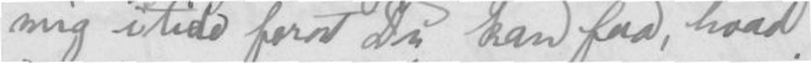mig i tide forat Du kan faa, hvad
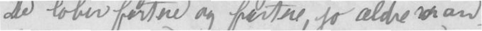De løber fortere og fortere, jo ældre man
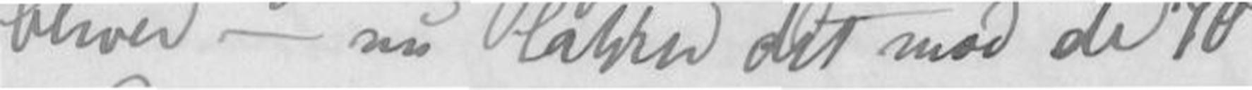bliver - nu lakker det mod de 70
